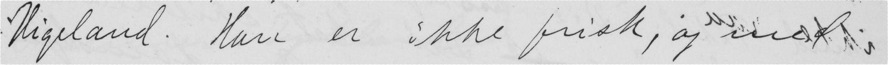Vigeland. Han er ikke frisk, og med
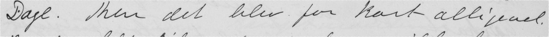Dage. Men det blev for kort alligevel.
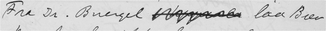Fra Dr. Buergel  laa Brev,
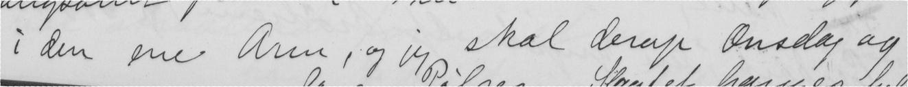i den ene Arm, og jeg skal derop Onsdag og
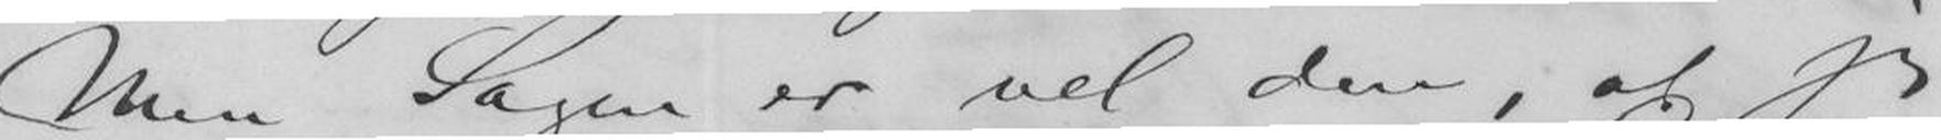Men Sagen er vel den, at jeg
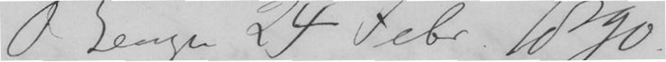Bergen 24 Febr. 1890
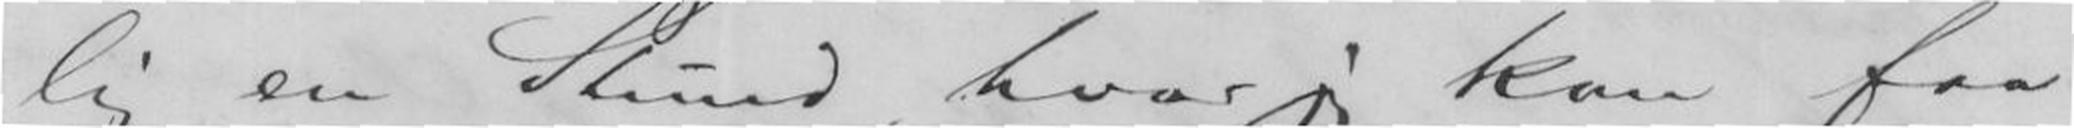lig en Stund, hvor jeg kan faa
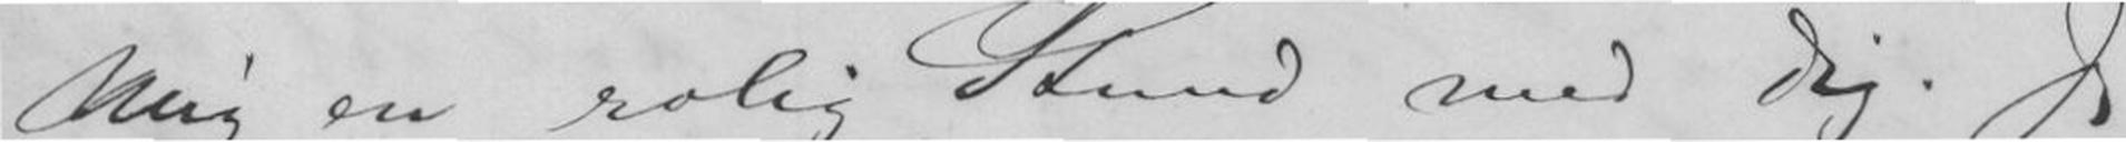Mig en rolig Stund med dig. Jeg
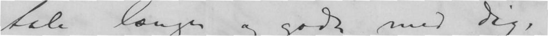tale længe og godt med dig, -
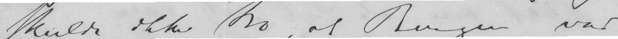skulde ikke tro, at Bergen var
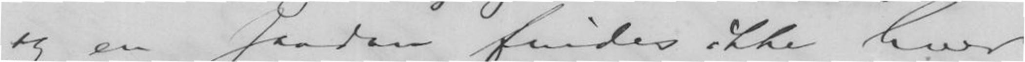og en saadan findes ikke hver
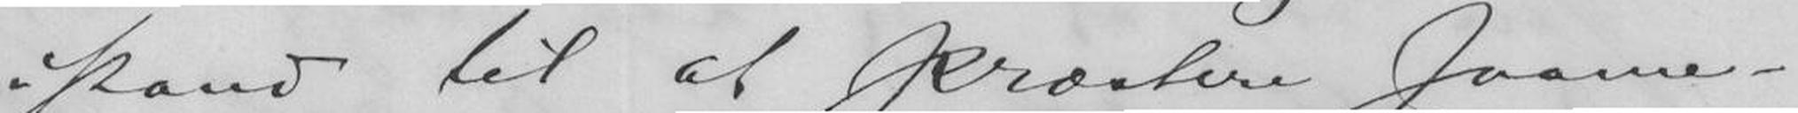istand til at præstere saame-
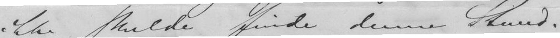ikke skulde finde denne Stund.
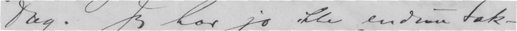Dag. Jeg har jo ikke endnu tak-
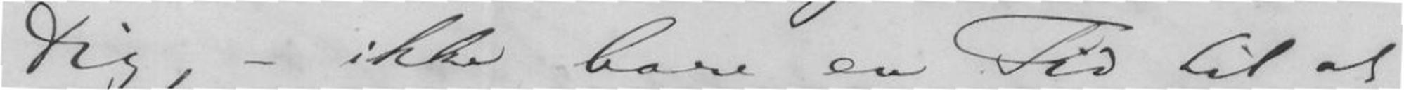Dig, - ikke bare en Tid til at
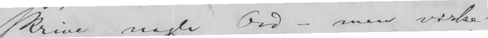skrive nogle Ord - men virke-
